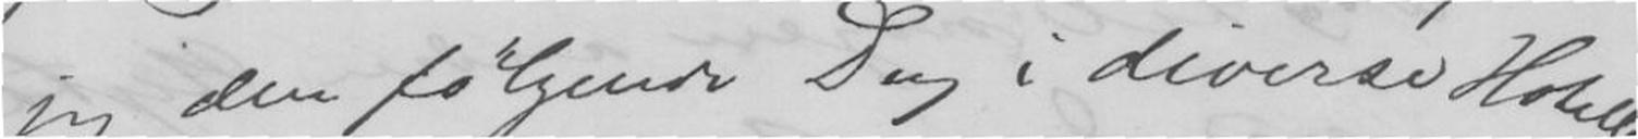jeg den følgende Dag i Diverse Hoteller
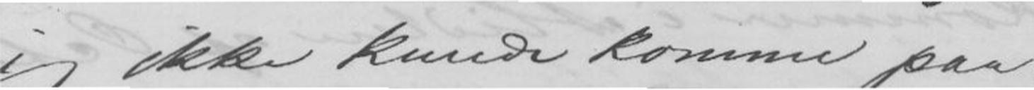jeg ikke kunde komme paa
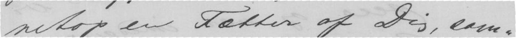netop en Fætter af Dig, sam-
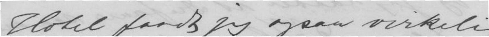Hotel fandt jeg ogsaa virkelig
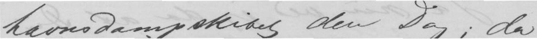havnsdampskibet den Dag; da
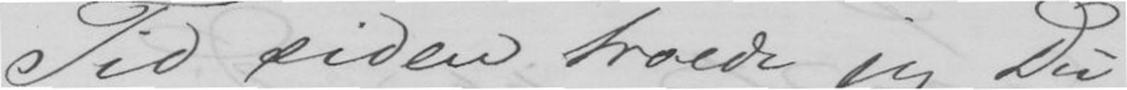Tid siden troede jeg Du
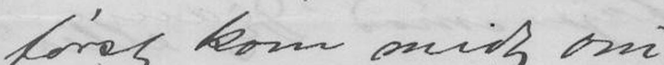først kom midt om
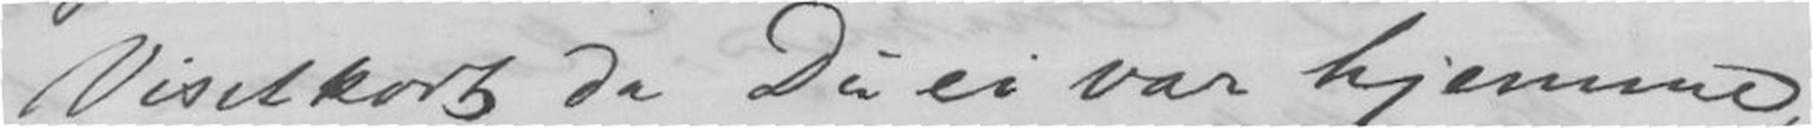Visitkort da Du ei var hjemme,
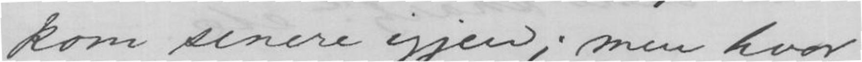kom senere igjen, men hvor
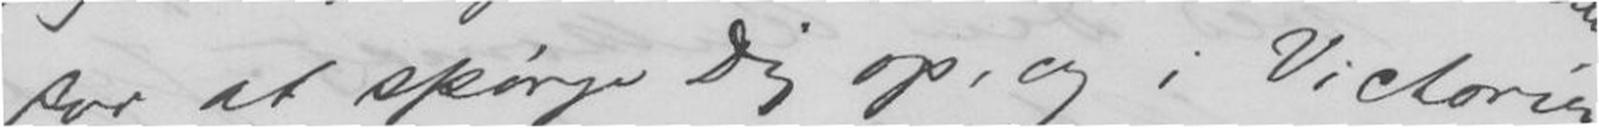for at spørge Dig op, og i Victoria
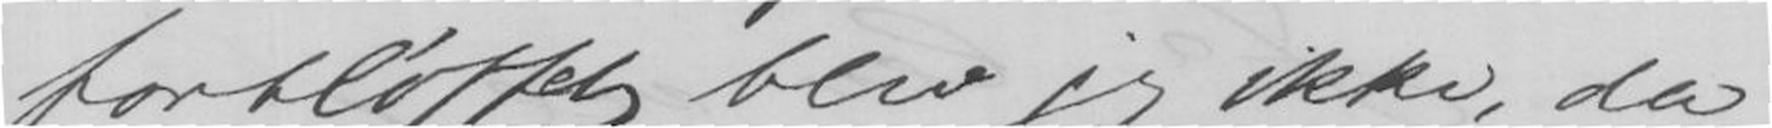forbløffet blev jeg ikke, da
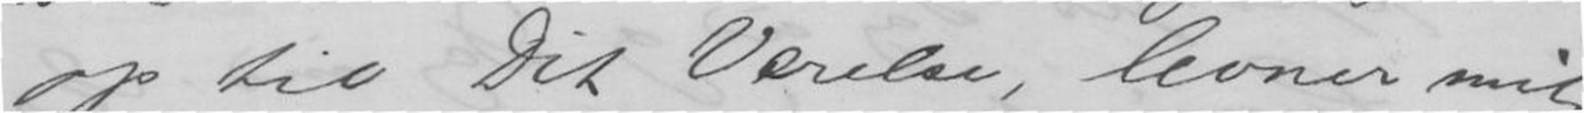op til Dit Værelse, levner mit
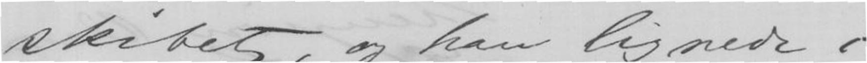skibet, og han lignede i
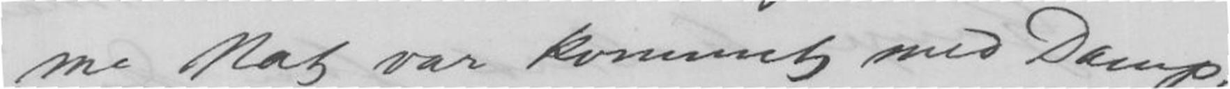me Nat var kommet med Damp
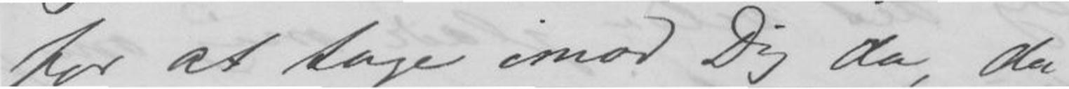for at tage imod Dig da, da
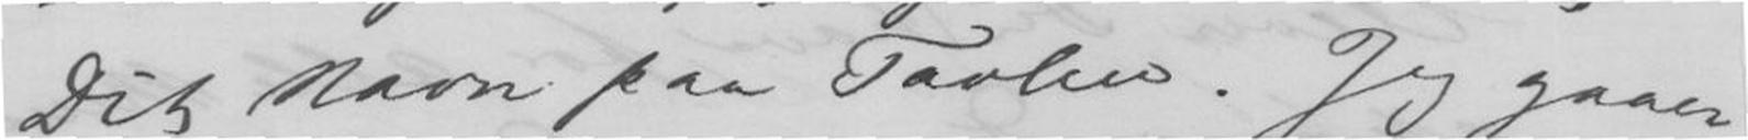Dit Navn paa Tavlen. Jeg gaar
